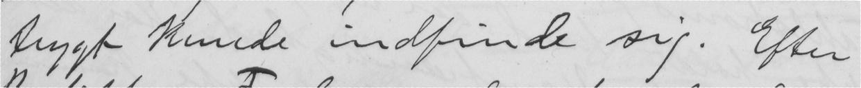trygt kunde indfinde sig. Efter
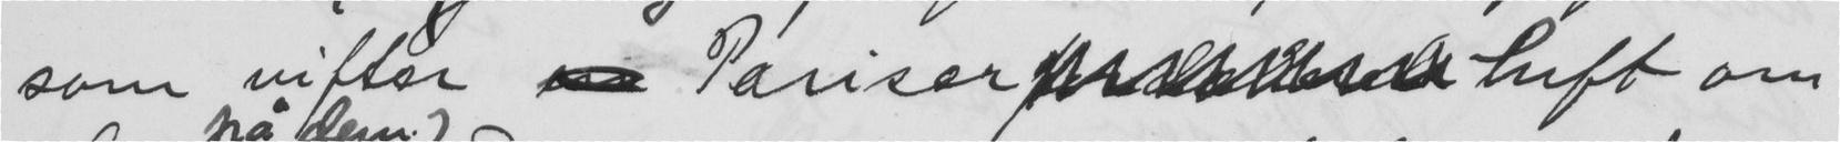som vifter  Pariser  tuft om
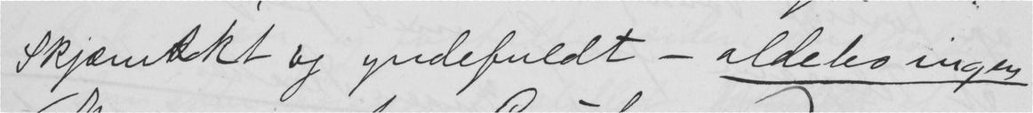Skjæmskt og yndefuldt — aldeles ingen.
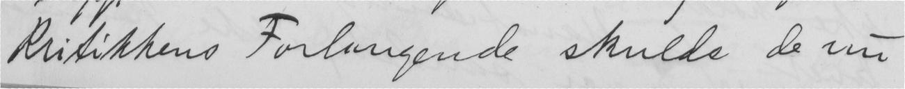Kritikkens Forlangende skulde de nu
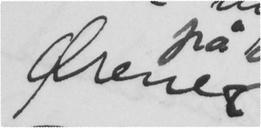Ørene
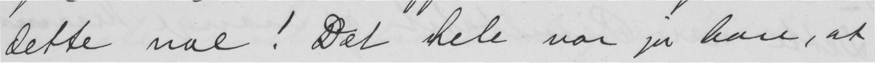dette noe. Det hele var jo bare, at
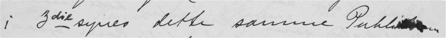i 3die synes dette samme Publikum
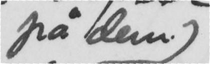på dem
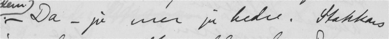- Da - jo mer jo bedre. Stakkars
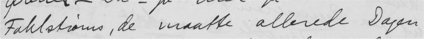Fahlstrøms, de maatte allerede Dagen
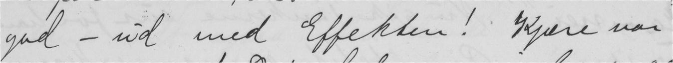god - ud med Effekten! Kjære var
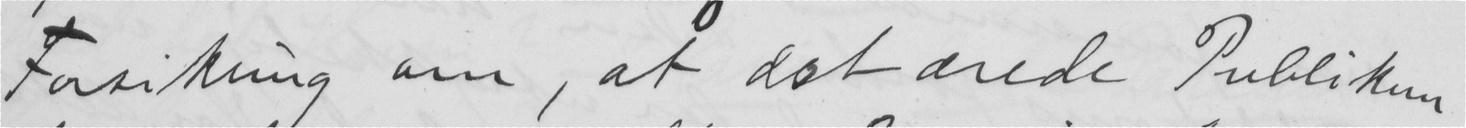Forsikning om at det ærede Publikum
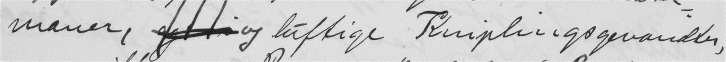maner,  og luftige Kniplingsgevandter,
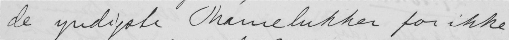de yndigste Mamelukker for ikke
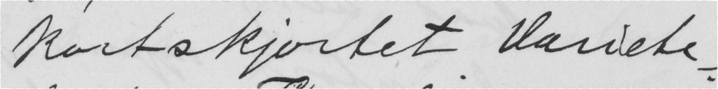kortskjørtet Variete-
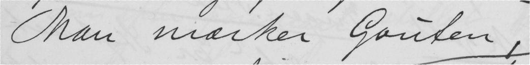Man mærker Gouten,
